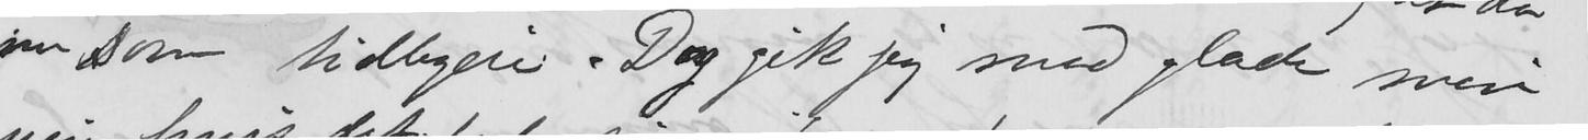nu som tidligere. Dog gik jeg med glæde min
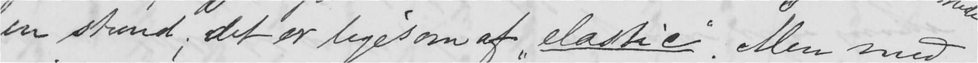en stund; det er ligesom af "elastic". Men med
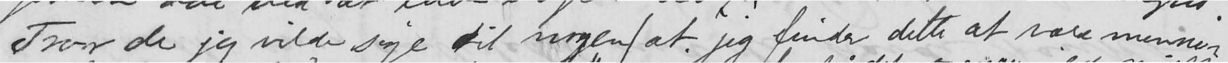Tror de jeg vilde sige til nogen at jeg finder dette at være menne-
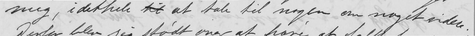mig, idethele til at tale til nogen om noget videre.
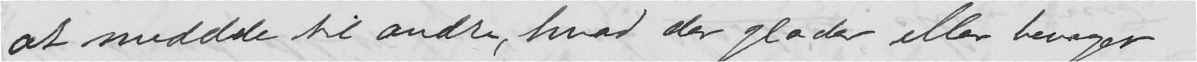at meddele til andre, hvad der glæder eller bevæger
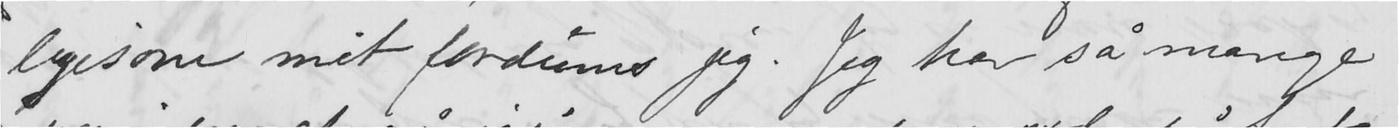ligesom mit fordums jeg. Jeg har så mange
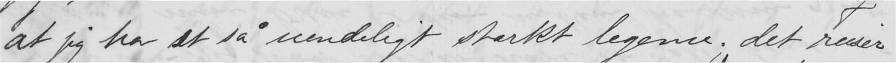at jeg har at så uendeligt sterkt legeme; det reiser
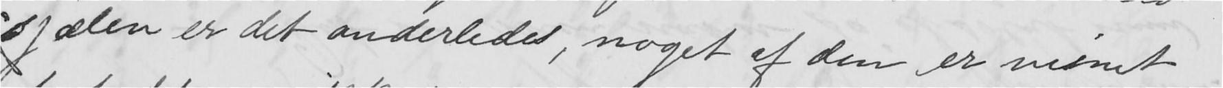sjælen er det anderledes, noget af den er visnet
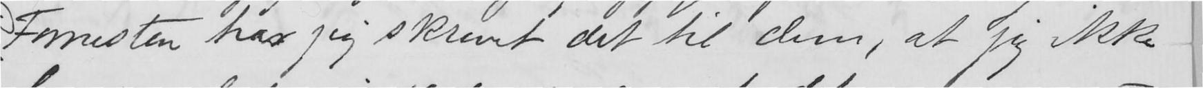Forresten har jeg skrevet det til dem, at jeg ikke
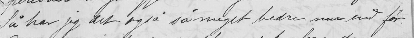Så har jeg det også så meget bedre nu end før.
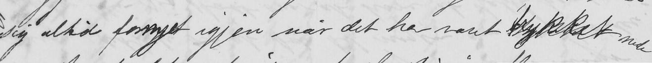sig altid fornyet igjen når det har været trykket nede
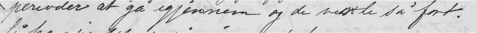perioder at gå igjennem og de vexle så fort.
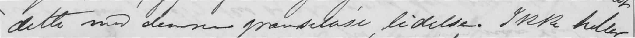dette med denne grænseløse lidelse. Ikke heller
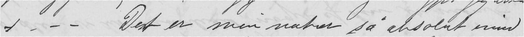- - - Det er min natur så absolut imod
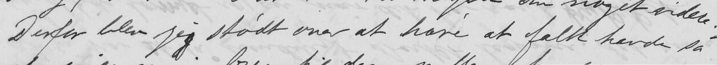Derfor blev jeg stødt over at høre at folk havde så
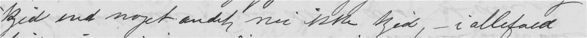kjed end noget andet, nei ikke kjed, - i allefald
